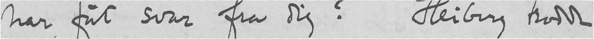har fåt svar fra dig? Heiberg trodde
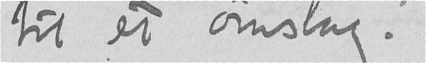til et omslag?
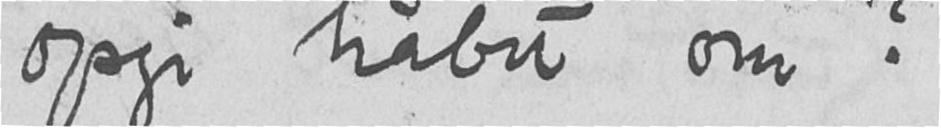opgi håbet om?
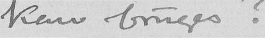kan bruges?
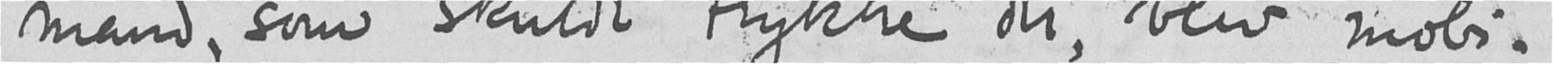mand, som skulde trykke det, blev mobi-
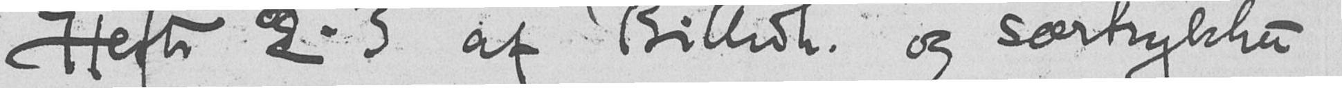Hefte 2-3 af Billedh. og særtrykket
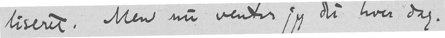liseret. Men nu venter jeg det hver dag.
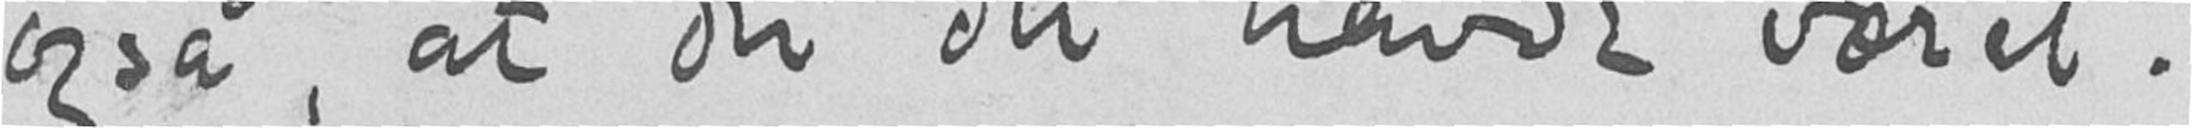også, at du det havde været.
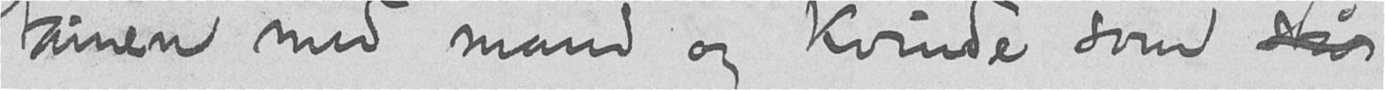tainen med mand og kvinde som står
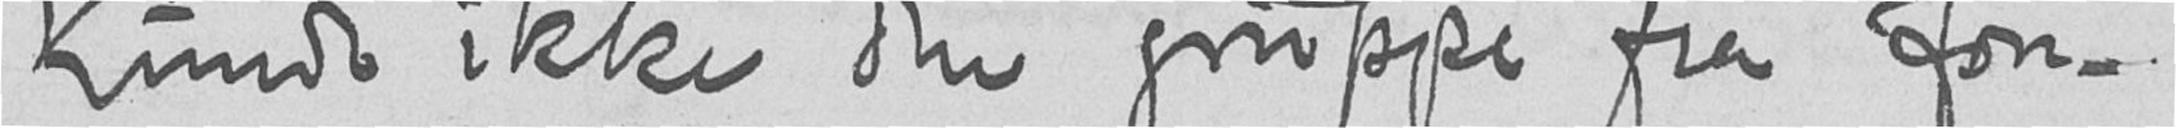Kunde ikke den gruppe fra fon-
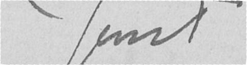Jens T
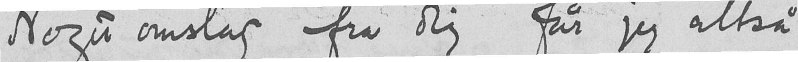Noget omslag fra dig får jeg altså
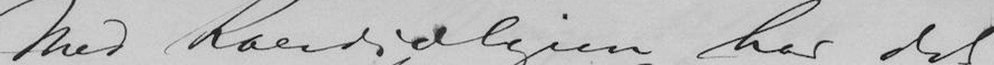Med Kardialgien har det
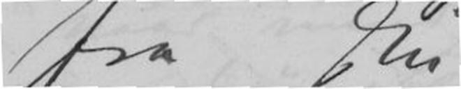fra Din
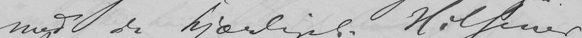med de kjærligste Hilsener
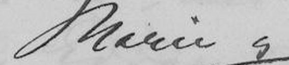Marie og
